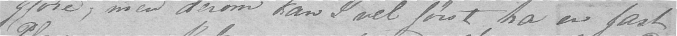gjøre, men derom kan I vel først ha er fast
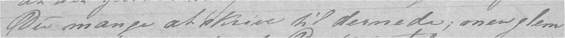Du mange at skrive til dernede; men glem
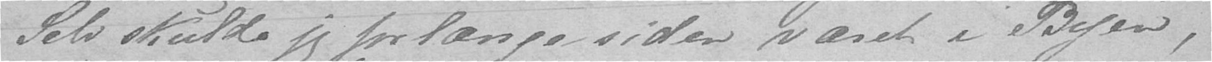Selv skulde jeg for længe siden været i Byen,
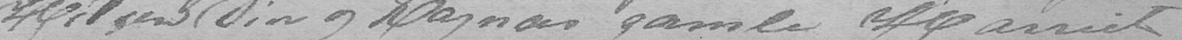Hilsen Din og Ragnas gamle Harriet
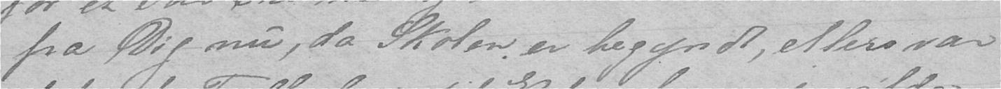fra Dig nu, da Skolen er begyndt, ellers var
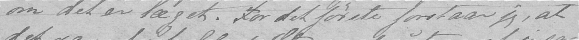om det er læget. For det første forstaar jeg, at
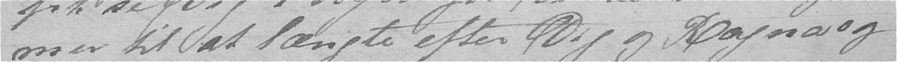men til at længte efter Dig og Ragna og
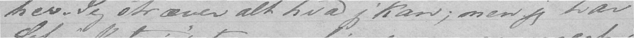her. Jeg stræver alt hvad jeg kan; men jeg har
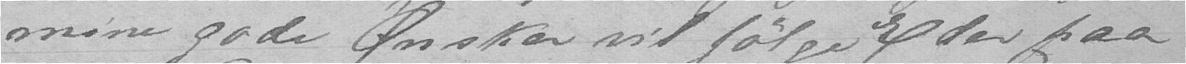mine gode Ønsker vil følge Eder paa
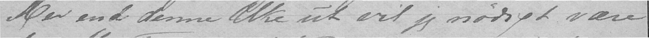Mer end denne Uke ut vil jeg nødigt være
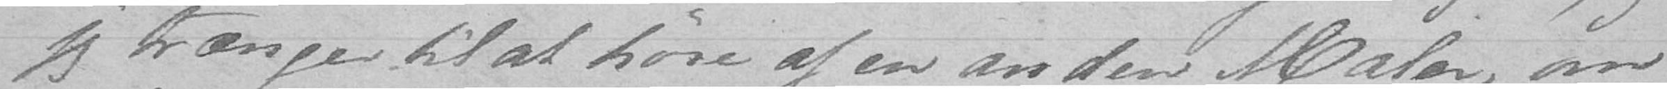jeg trænger til at høre af en anden Maler, om
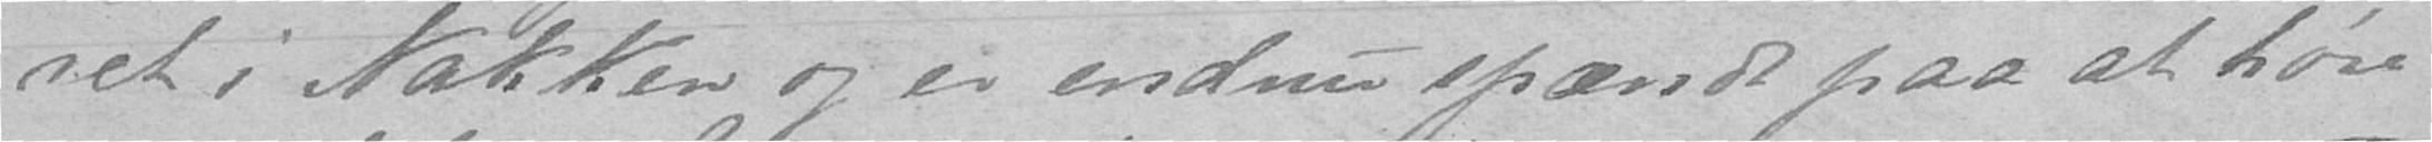ret i Nakken og er endnu spændt paa at høre
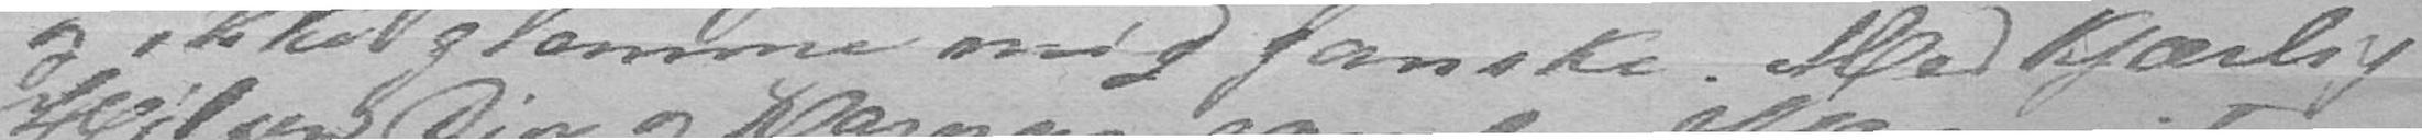og ikke glemme mig ganske. Med Kjærlig
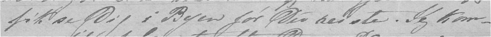fik se Dig i Byen for Du reiste. Jeg kom-
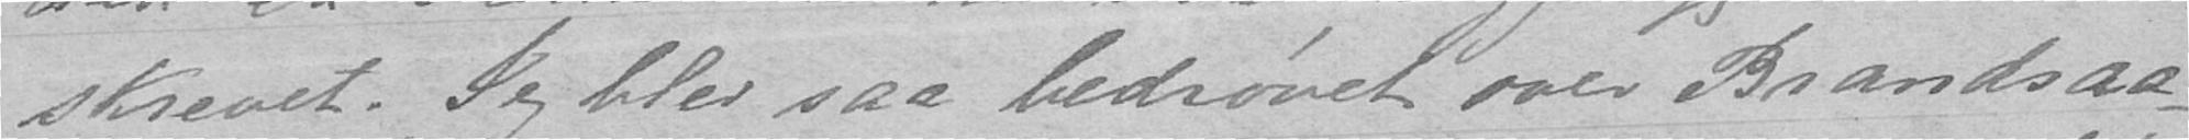skrevet. Jeg blev saa bedrøvet over Brandsaa-
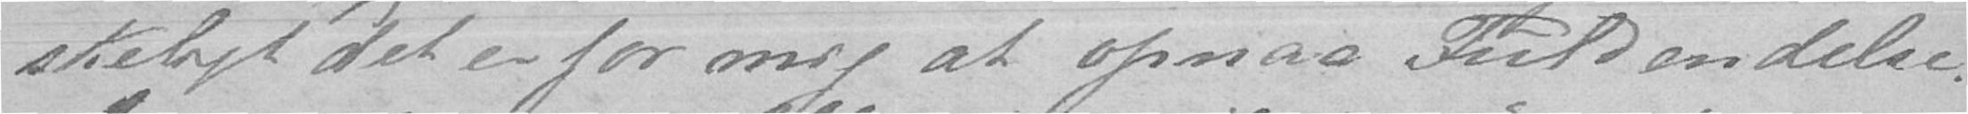skeligt det er for mig at opnaa Fuldendelse.
